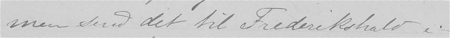men send det til Frederikshald i-
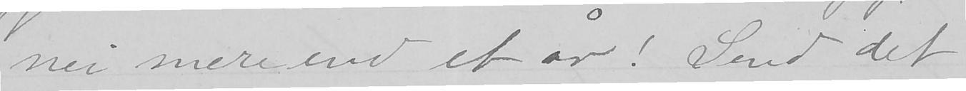nei mere end et år! Send det
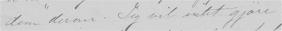dem derom. Jeg vil intet gjøre
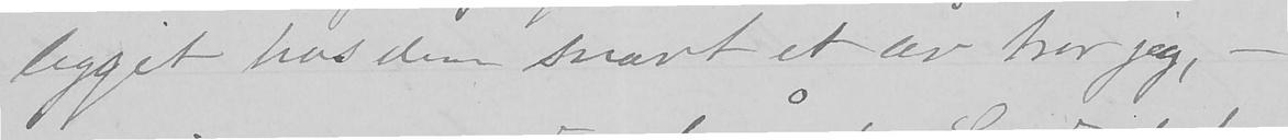ligget hos dem snart et år tror jeg, -
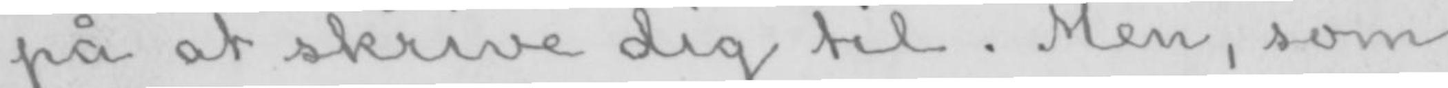på at skrive dig til. Men, som
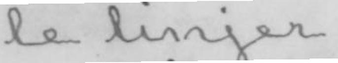le linjer.
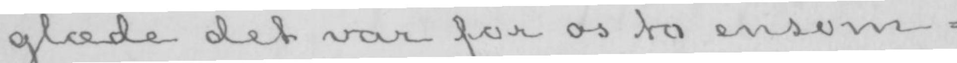glæde det var for os to ensom-
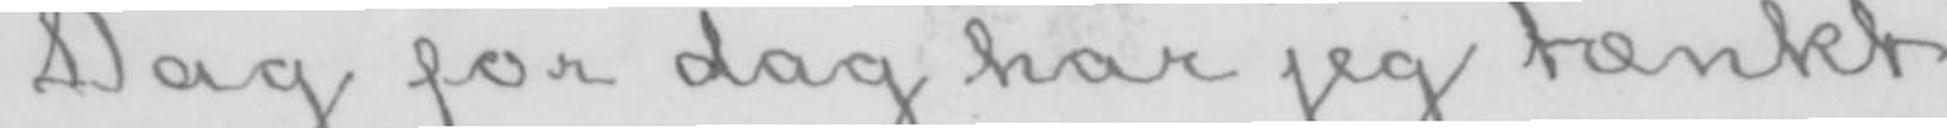Dag for dag har jeg tænkt
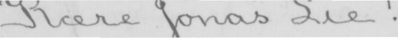Kære Jonas Lie!
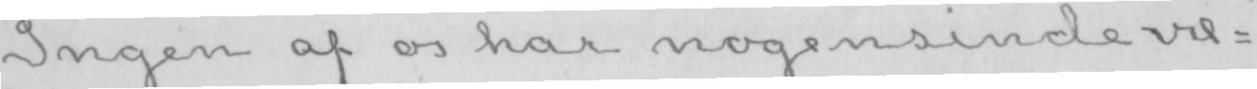Ingen af os har nogensinde væ-
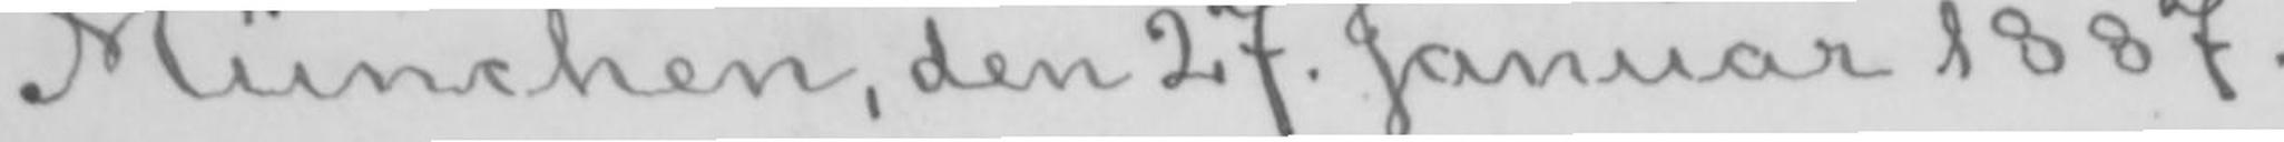München, den 27. Januar 1887.
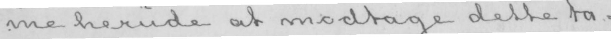me herude at modtage dette ta-
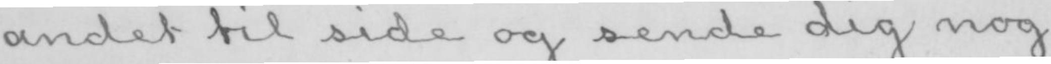andet til side og sende dig nog-
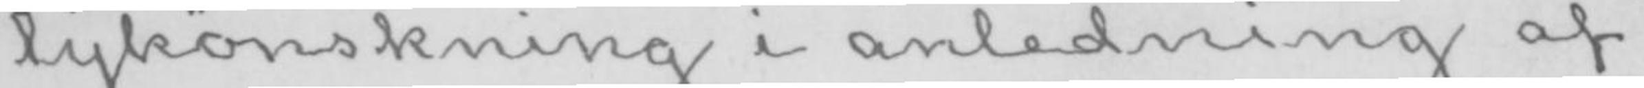lykønskning i anledning af
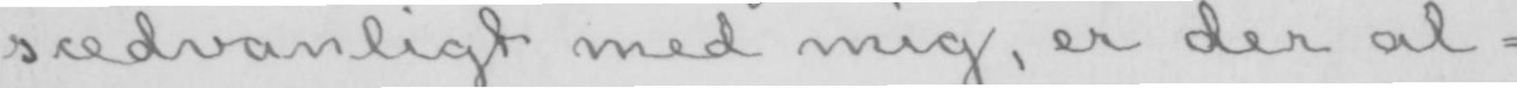sædvanligt med mig, er der al-
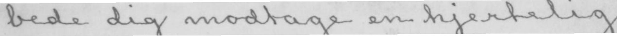bede dig modtage en hjertelig
